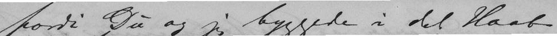fordi Du og jeg byggede i det Haab
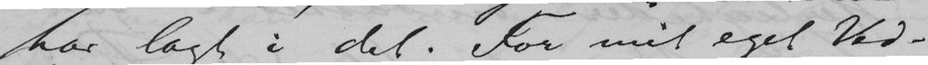har lagt i det. For mit eget Ved-
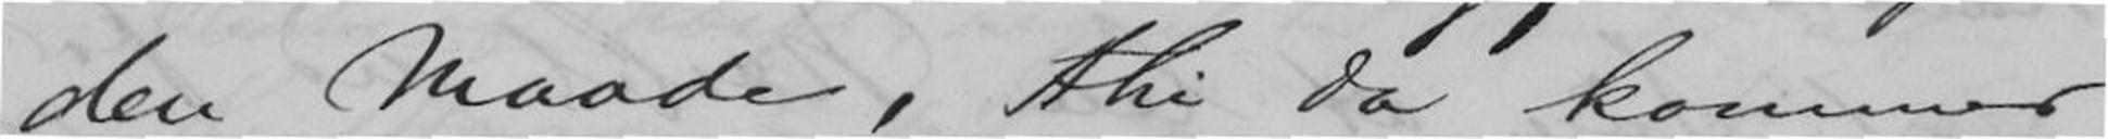den Maade, thi da kommer
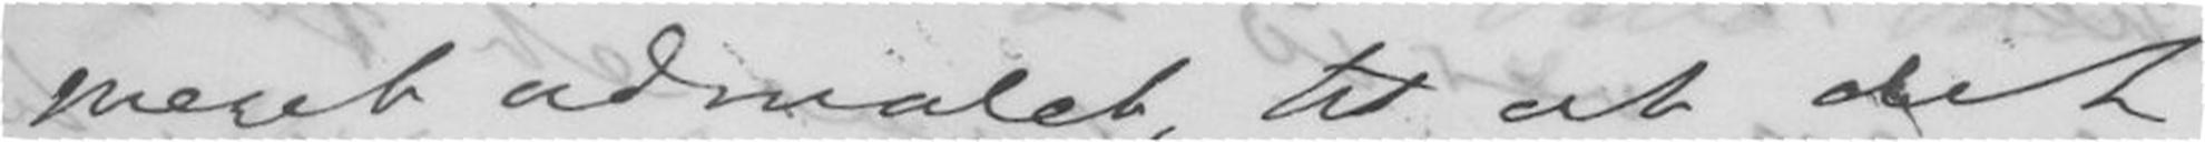meget udmalet, til at det
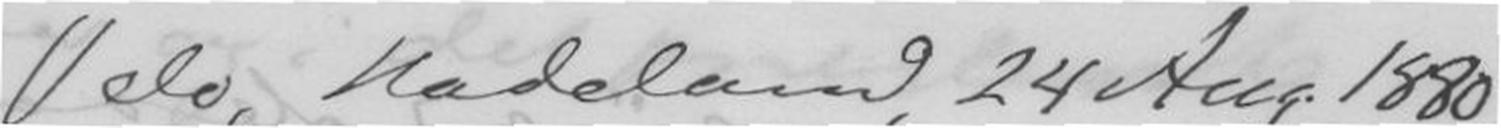Velo, Hadeland, 24 Aug. 1880
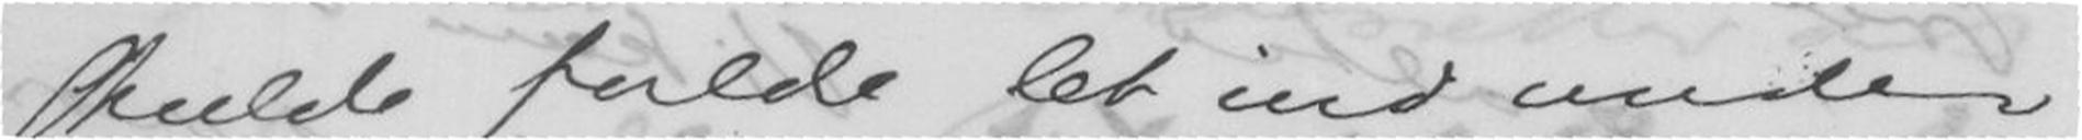skulde falde let ind under
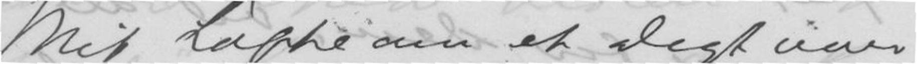Mit Løfte om et Digt over
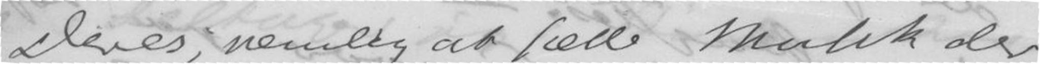Deres, nemlig at sætte Musik der-
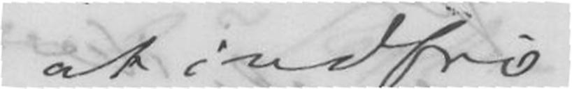at indfri
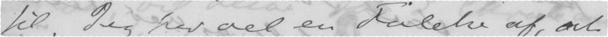til. Jeg har vel en Følelse af, at
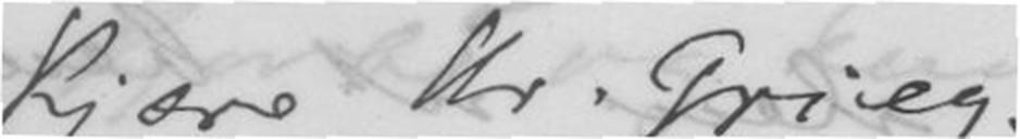Kjære Hr. Grieg.
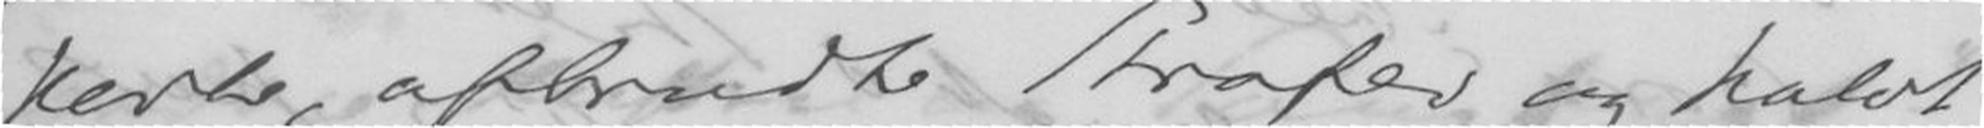kendte, afbrudte Strofer og halvt
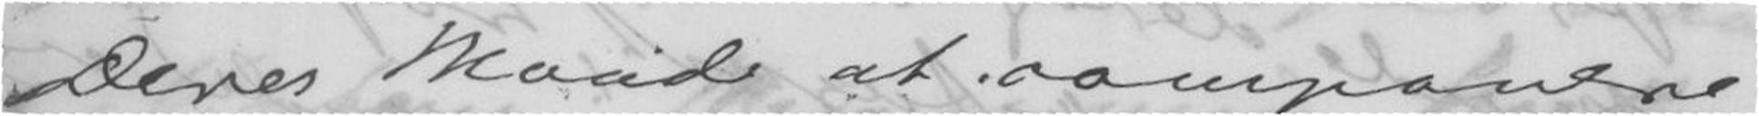Deres Maade at componere
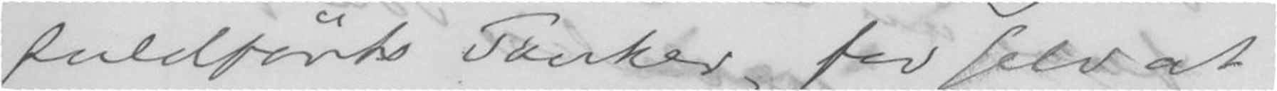fuldførte Tanker, for selv at
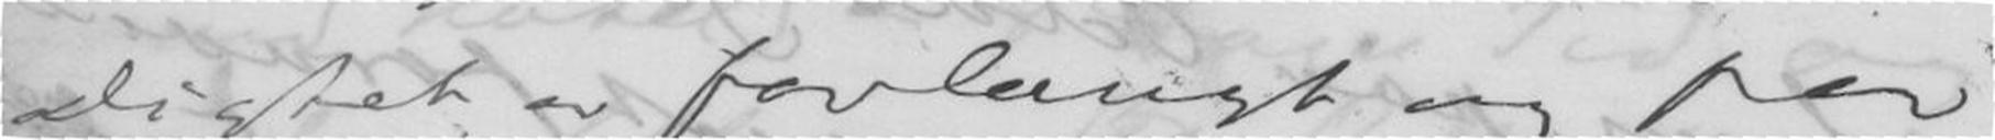Digtet er forlangt og for
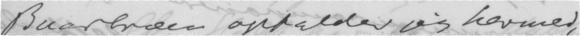Buarbræen opfylder jeg hermed,
